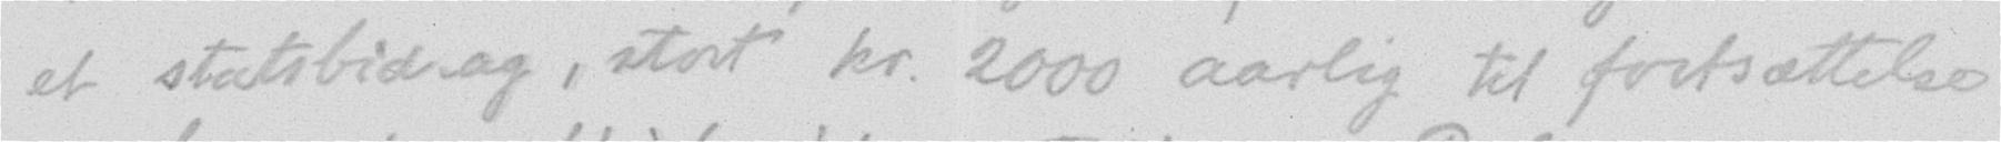et statsbidrag, stort kr. 2000 aarlig til fortsættelse
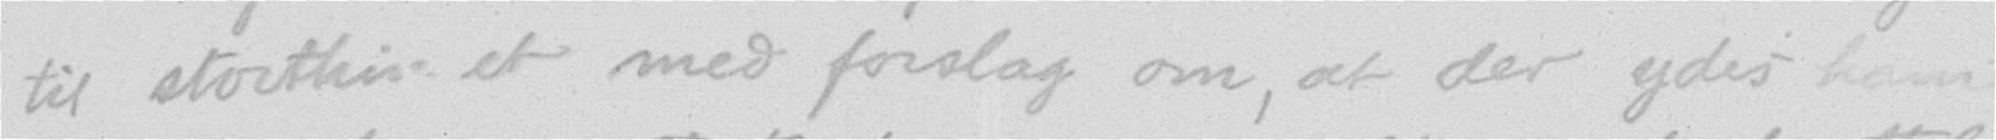til storthinget med forslag om, at der ydes ham
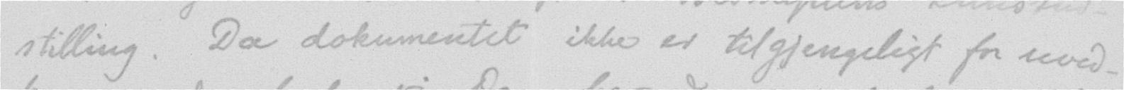stilling. Da dokumentet ikke er tilgjengeligt for uved-
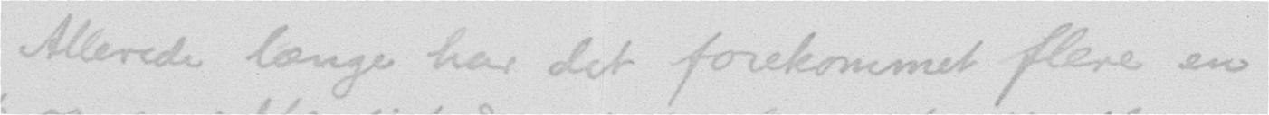Allerede længe har det forekommet flere en
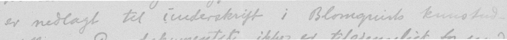er nedlagt til underskrift i Blomquists kunstud-
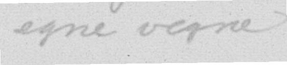egne vegne
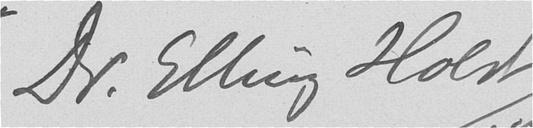Dr. Elling Holst
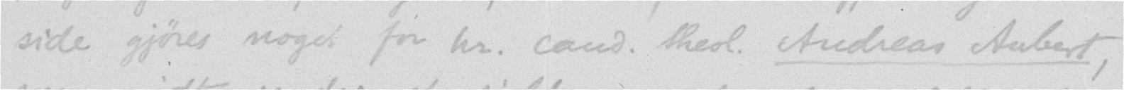side gjøres noget for hr. cand. theol. Andreas Aubert,
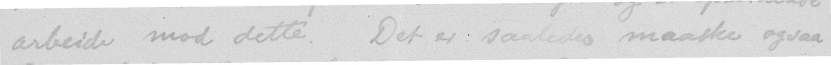arbeide mod dette. Det er saaledes maaske ogsaa
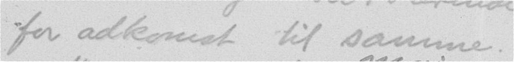for adkomst til samme.
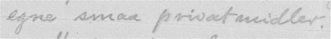egne smaa privatmidler.
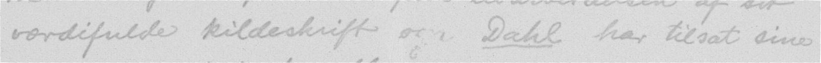værdifulde kildeskrift om Dahl har tilsat sine
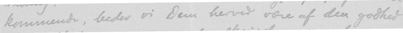kommende, beder vi Dem herved være af den godhed
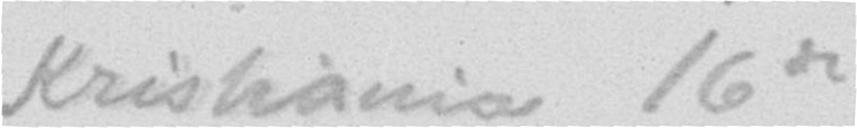Kristiania 16de
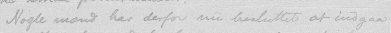Nogle mænd har derfor nu besluttet at indgaa
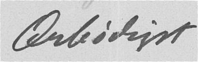Ærbødigst
